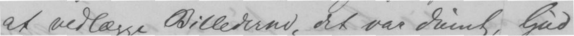at vedlægge Billederne, det var dumt, Gud
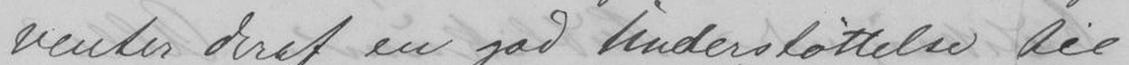venter deraf en god understøttelse til
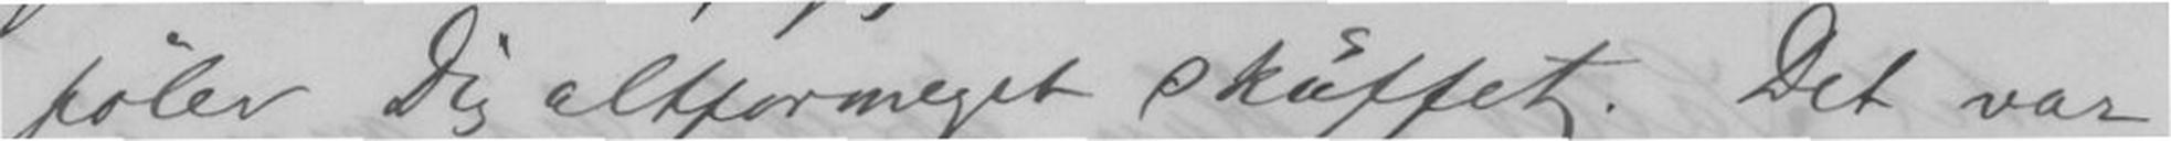føler Dig altformeget skuffet. Det var
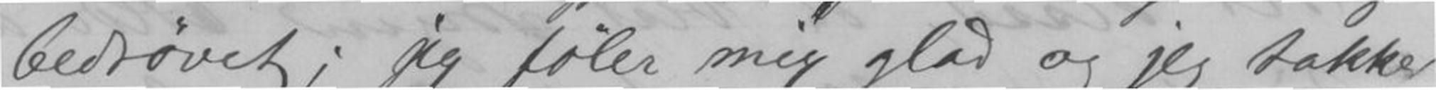bedrøvet, jeg føler mig glad og jeg takker
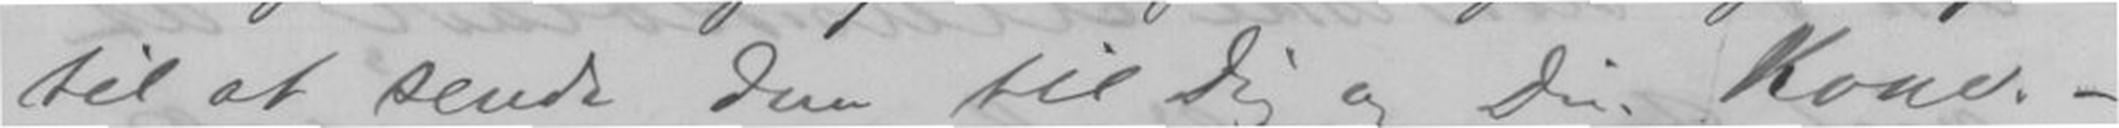til at sende Dem til Dig og Din Kone.-
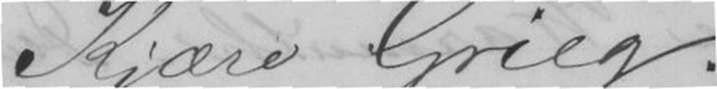Kjære Grieg!
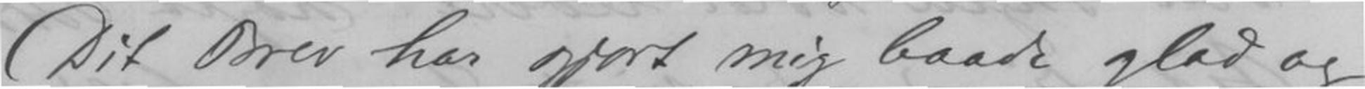Dit Brev har gjort mig baade glad og
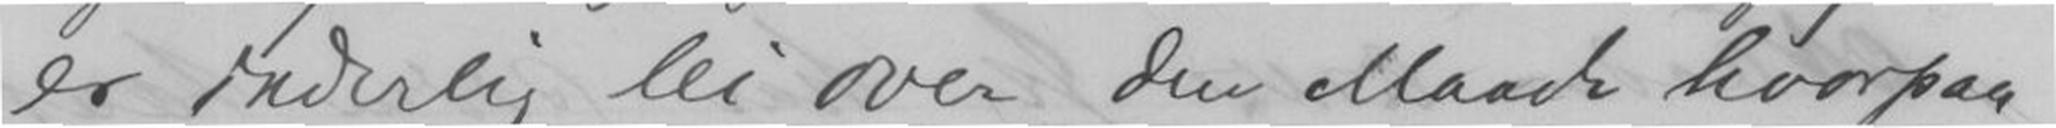er inderlig lei over den Maade hvorpaa
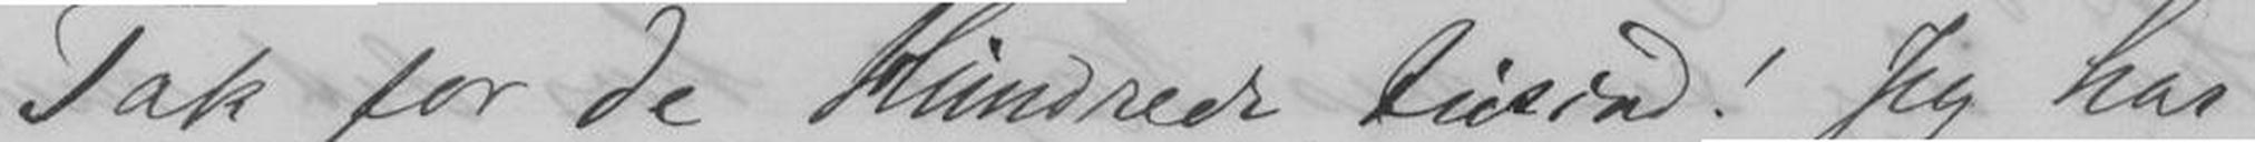Tak for de Hundrede tusind! Jeg har
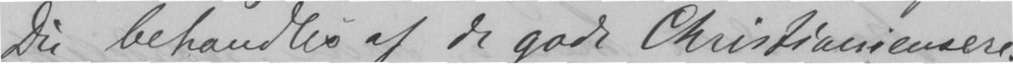Du behandles af De gode Christianiensere.
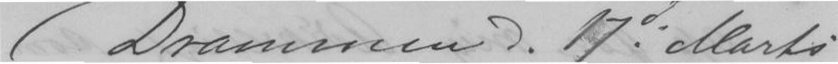Drammen d. 17d Marts
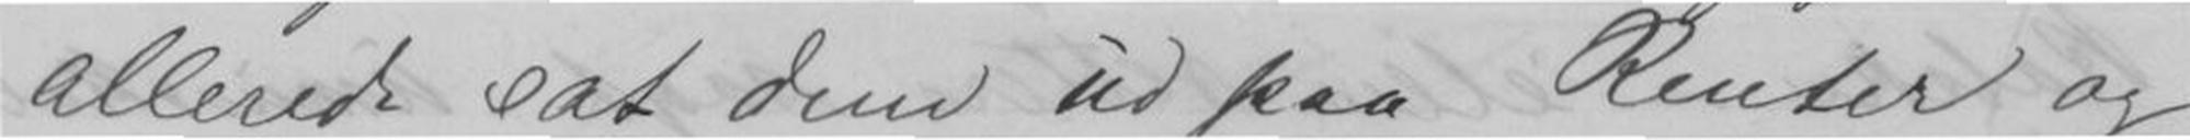allerede sat dem ud paa Renter og
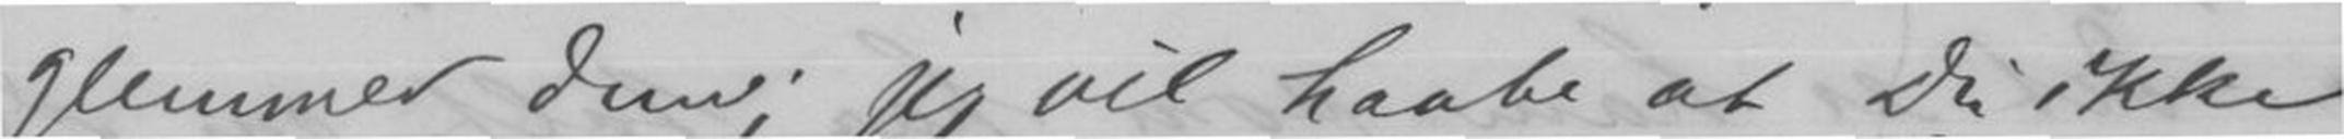glemmer dem; jeg vil haabe at Du ikke
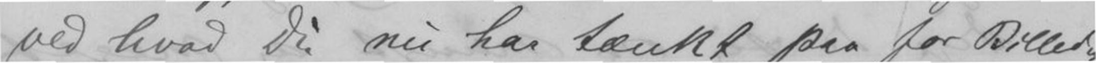ved hvad Du nu har tænkt paa for Billed,
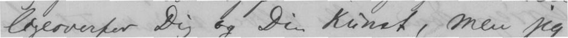ligeoverfor Dig og Din Kunst, men jeg
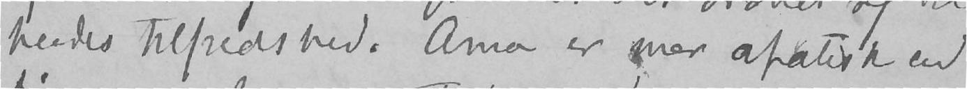hendes tilfredshed. Anna er mer apatisk end
